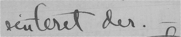senteret der. -
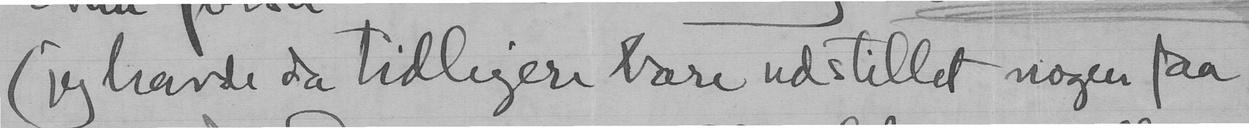(jeg havde da tidligere bare udstillet nogen faa
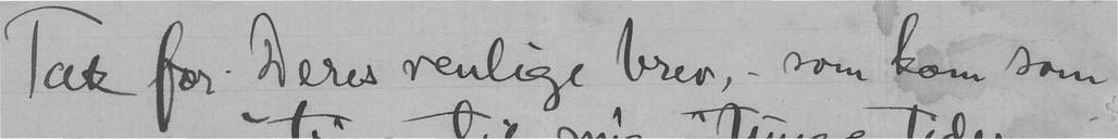Tak for Deres venlige brev, - som kom som
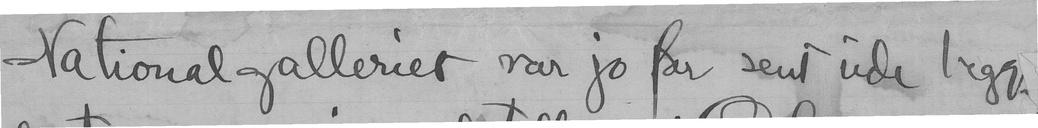Nationalgalleriet var jo for sent ude begge
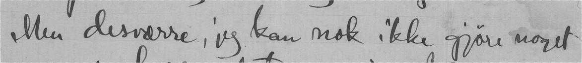Men desværre, jeg kan nok ikke gjøre noget
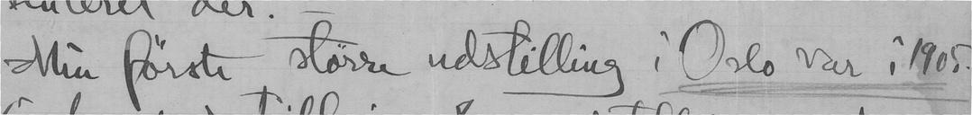Min første større udstilling i Oslo var i 1905
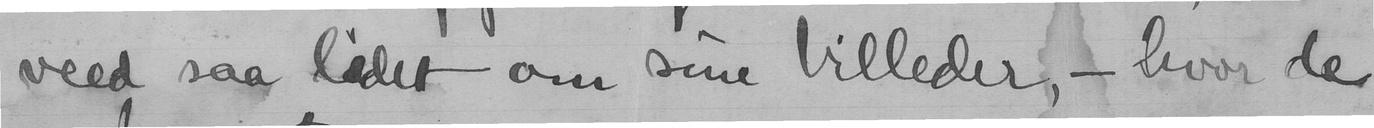veed saa lidet om sine billeder, - hvor de
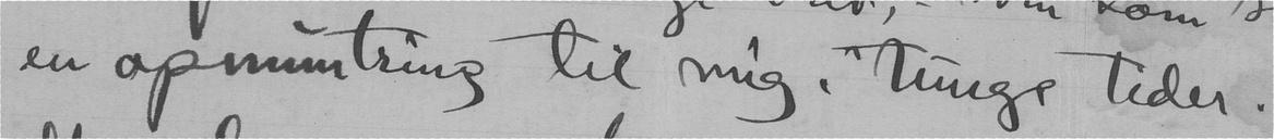en opmuntring til mig i tunge tider.
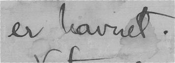er havnet.
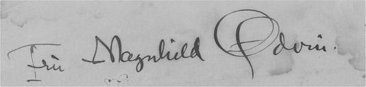Fru Magnhild Ødvin.
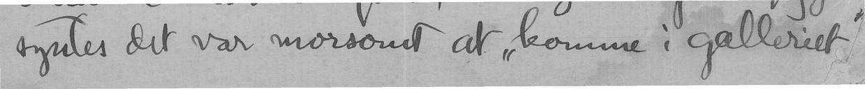syntes det var morsomt at "komme i galleriet"
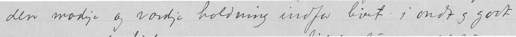den modige og værdige holdning indfor livet i ondt og godt
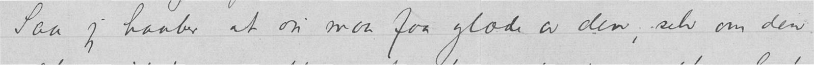Saa jeg haaber at du maa faa glæde av den, selv om den
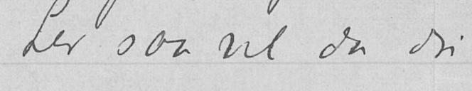Lev saa vel da du
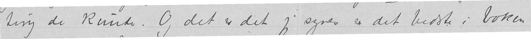ting de kunde. Og det er det jeg synes er det bedste i boken
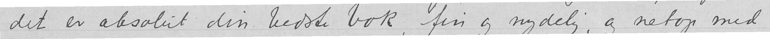det er absolut din bedste bok, fin og nydelig, og netop med
